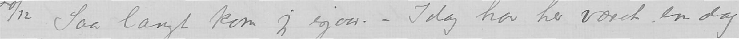20/12 Saa langt kom jeg igaar. - Idag har her været en dag
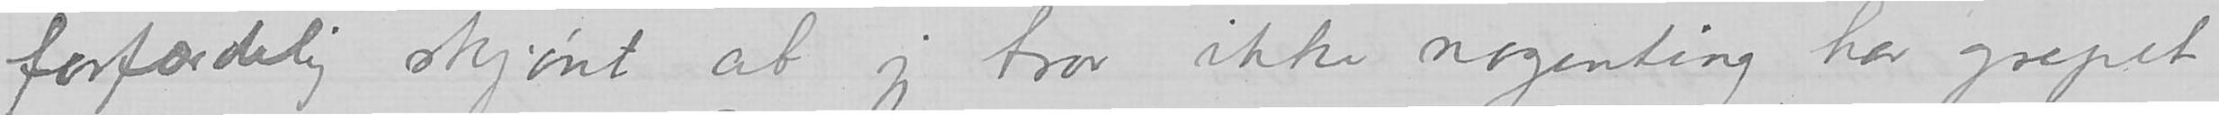forfærdelig skjønt at jeg tror ikke nogenting har grepet
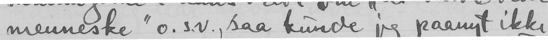menneske" o.s.v., saa kunde jeg paanyt ikke
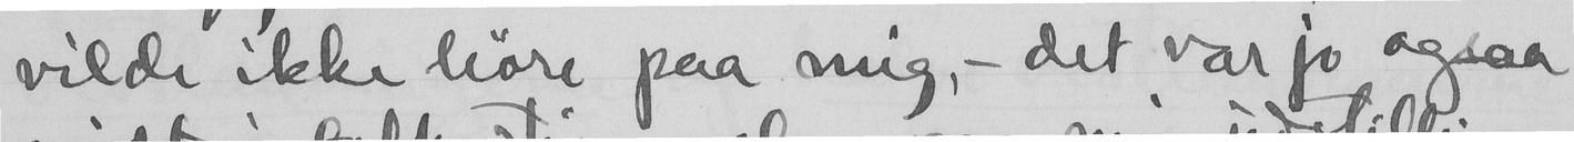vilde ikke høre paa mig, - det var jo ogsaa
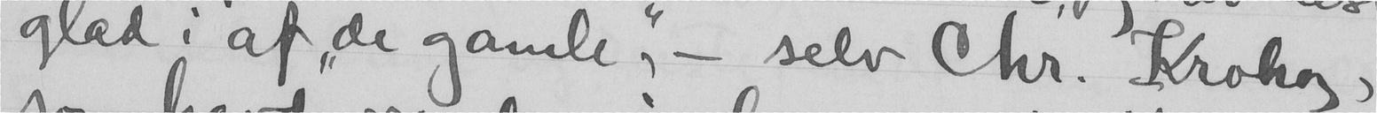glad i af "de gamle, - selv Chr. Krohg,
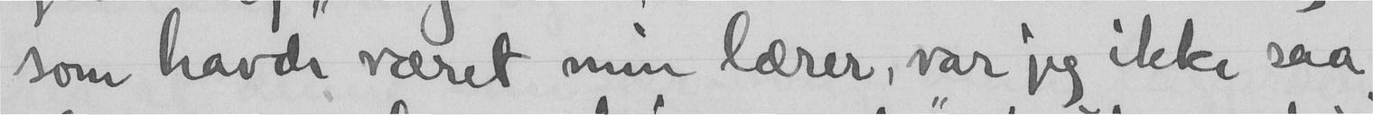som havde været min lærer, var jeg ikke saa
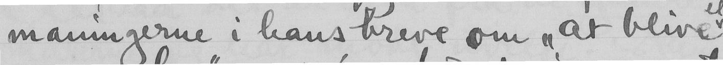maningerne i hans breve om "at blive
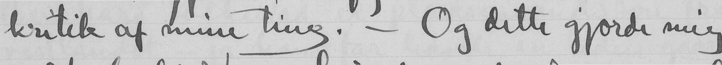kritik af mine ting. - Og dette gjorde mig
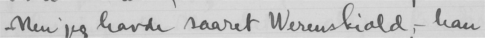Men jeg havde saaret Werenskiold, - han
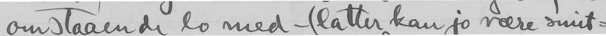omstaaende lo med - (latter kan jo være smit-
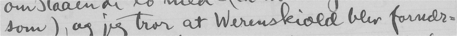som), og jeg tror at Werenskiold blev fornær-
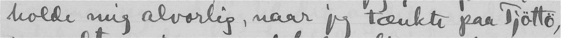holde mig alvorlig, naar jeg tænkte paa Tjøttø,
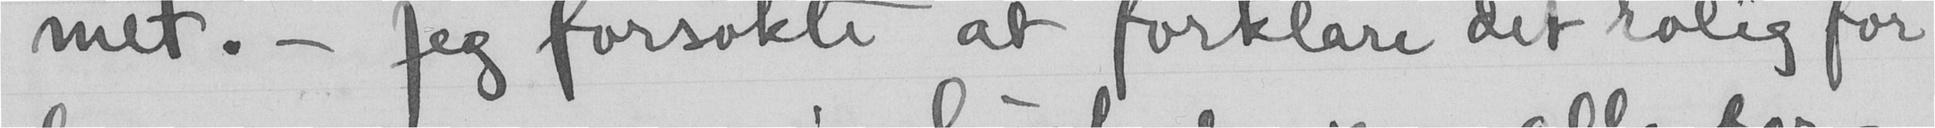met. - Jeg forsøkte at forklare det rolig for
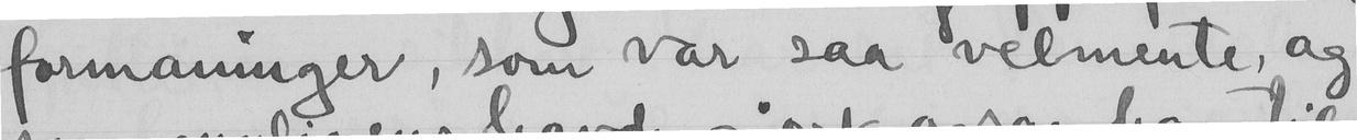formaninger, som var saa velmente, og
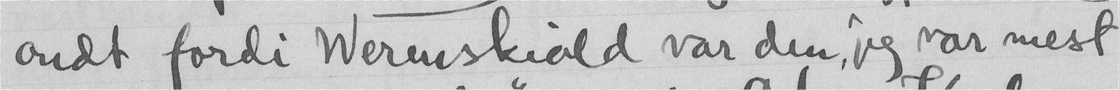ondt fordi Werenskiold var den, jeg var mest
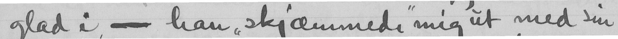glad i - han "skjæmmede" mig ut med sin
